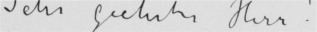Sehr geehrter Herr!
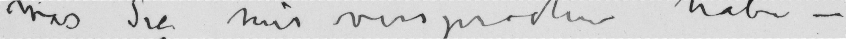was Sie mir versprochen habe –
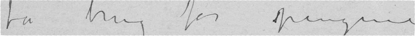få brug for pengene i
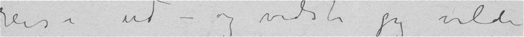reise ud – og vidste jeg vilde
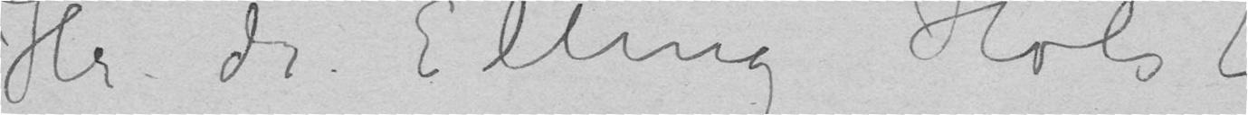Hr. dr. Elling Holst
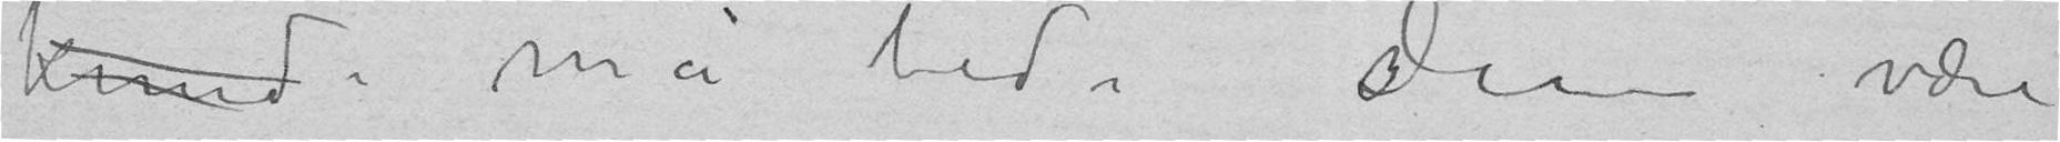kund må bede Dem være
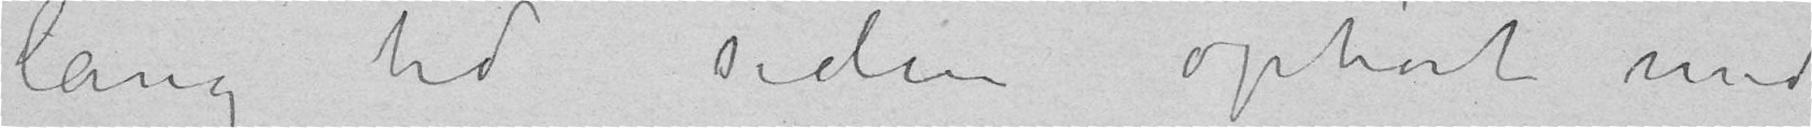lang tid siden ophørt med
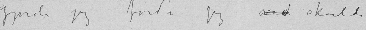gjorde jeg fordi jeg ved skulde
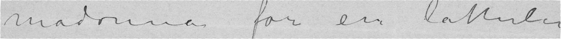Madonna for en latterlig
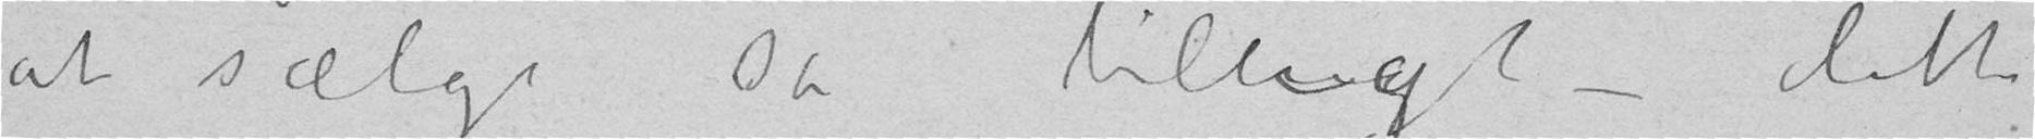at sælge så billigt – dette
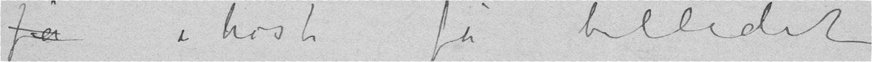få i høst få billedet
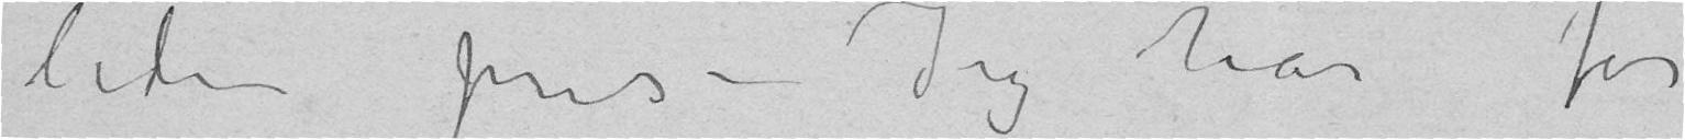liden pris – Jeg har for
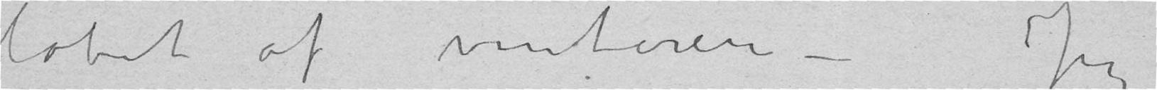løbet af vinteren – Jeg
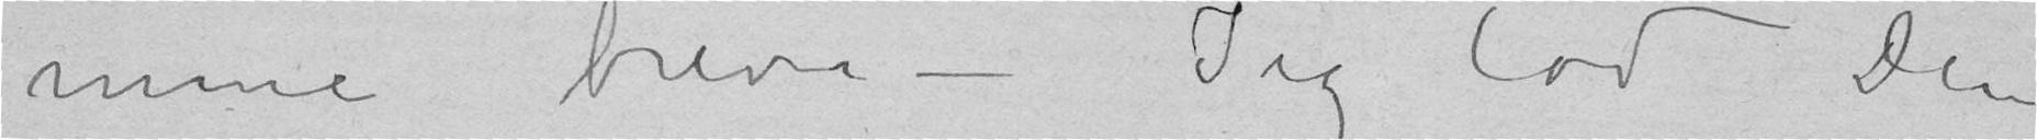mine breve – Jeg lod Dem
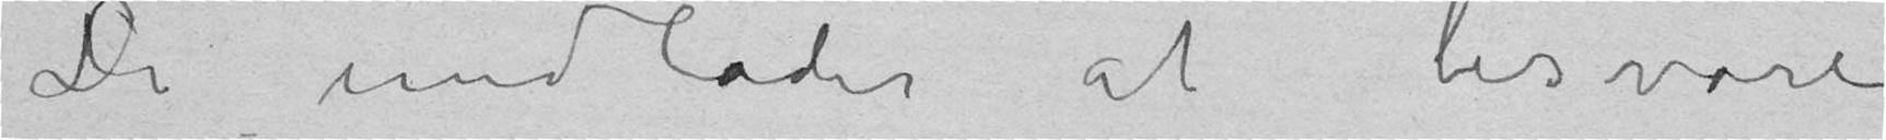De undlader at besvare
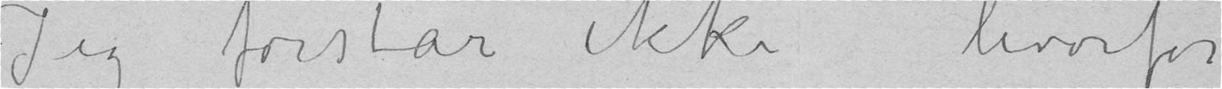Jeg forstår ikke hvorfor
